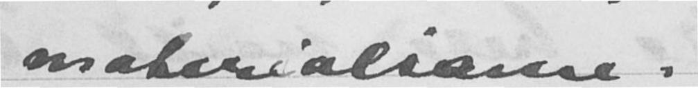og materialisme.
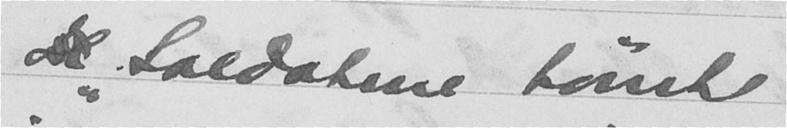"Soldatene tømte
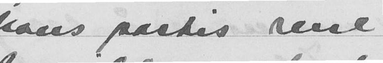hans partis rene
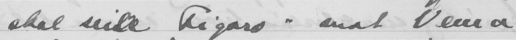skal seile "Figaro" mot Vema
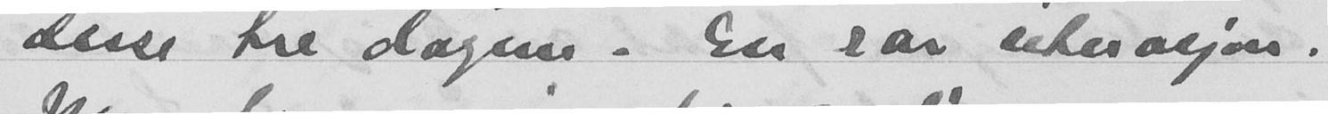disse tre dagen. En rar situasjon
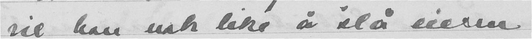vil han nok like å slå eieren
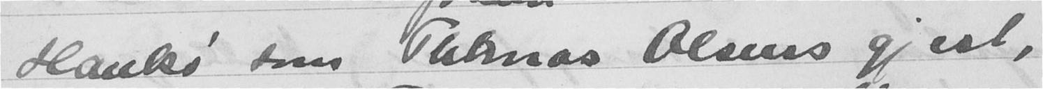Hankø som Thomas Olsens gjest,
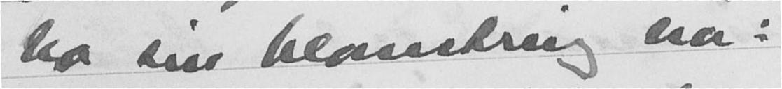la sin blomstring nå:
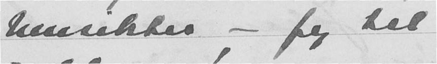hensikter - fy til
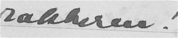rakkeren.
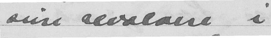sin revalæise i
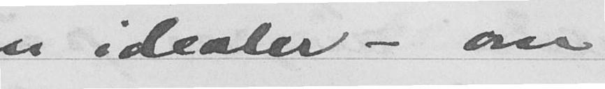om idealer - om
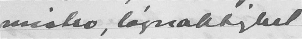mistro, løgnaktighet
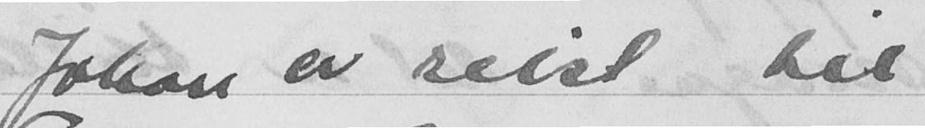Johan er reist til
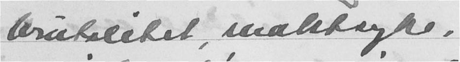brutalitet, maktsyke,
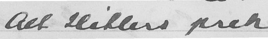Alt Hitlers prat
